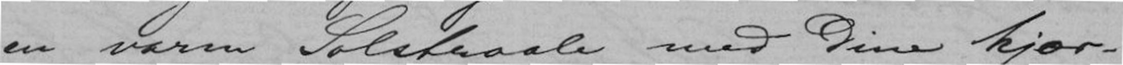en varm Solstråle med Dine kjær-
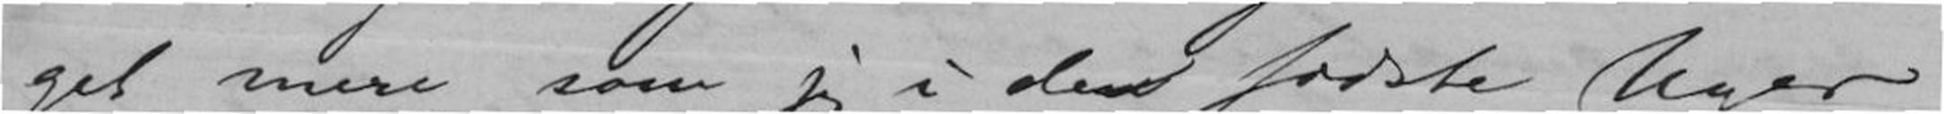get mere som jeg i de "n" i "den" sidste Uger
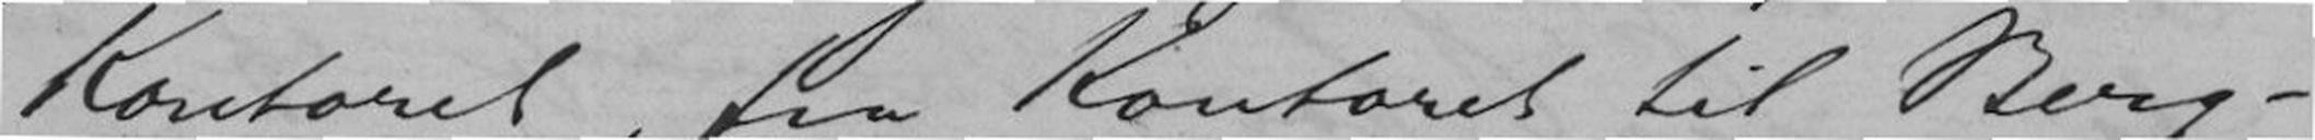Kontoret, fra Kontoret til Berg-
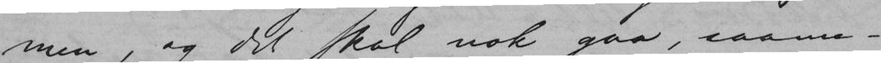men, og det skal nok gaa, saame-
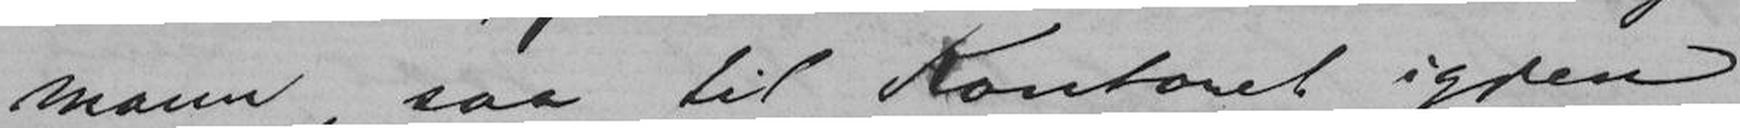mann, saa til Kontoret igjen
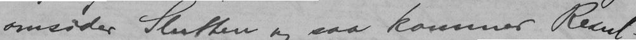omsider Slutten og saa kommer Resul-
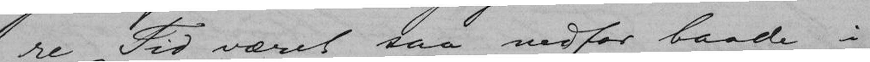re Tid været saa nedfor baade i
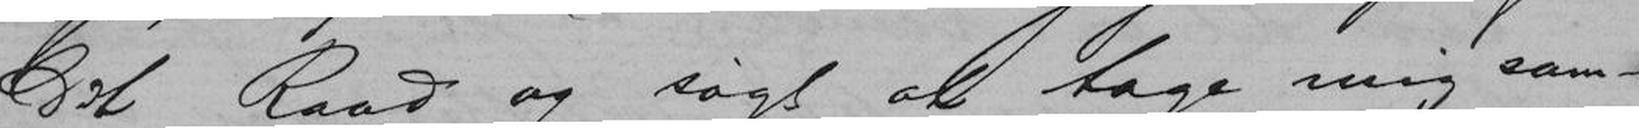Dit Raad og søgt at tage mig sam-
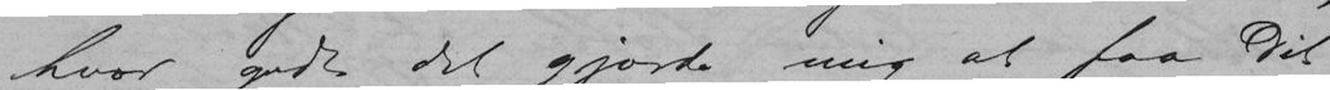hvor godt det gjorde mig at faa Dit
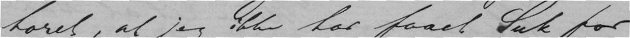toret, at jeg ikke har faaet Suk for
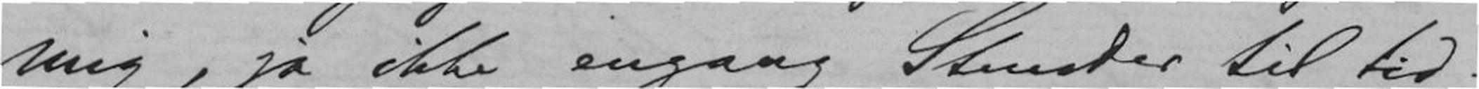mig, ja ikke engang Stunder til tid-
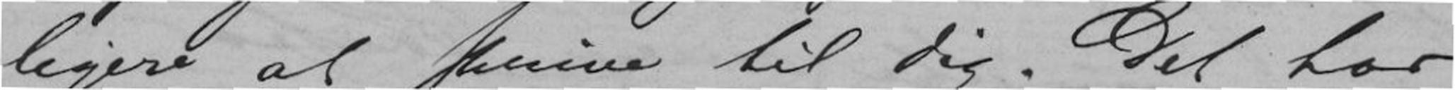ligere at skrive til dig. Det har
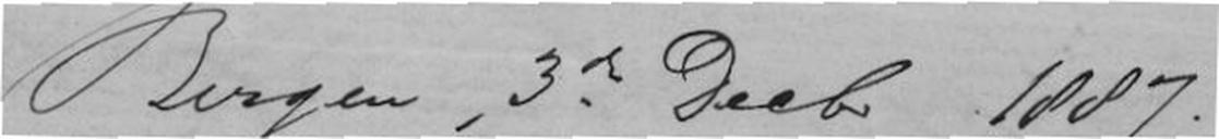Bergen, 3die Decb 1887
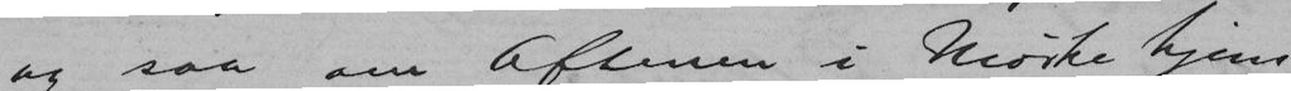og saa om Aftenen i Mørke hjem
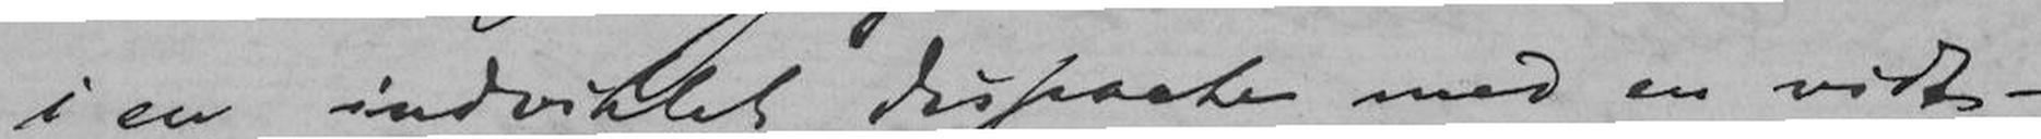i en indviklet dispache med en vidt-
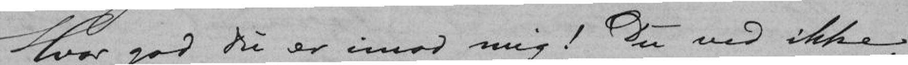Hvor god du er imod mig! Du ved ikke,
